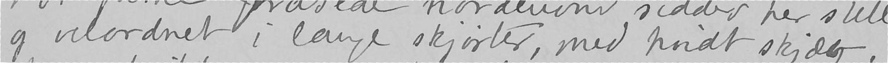og velordnet i lange skjørter, med hvidt skjæg,
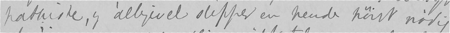pathiske, og alligevel slipper en hende høist nødig
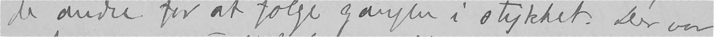de andre for at følge gangen i stykket. Der var
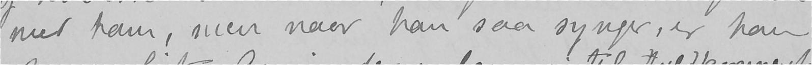med ham, men naar han saa synger, er han
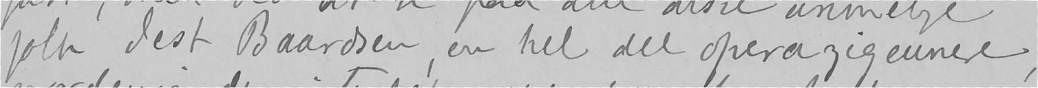folk Jest Baardsen, en hel del operazigeunere,
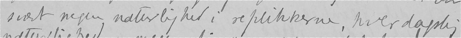svært megen naturlighed i replikkerne, hverdagslig
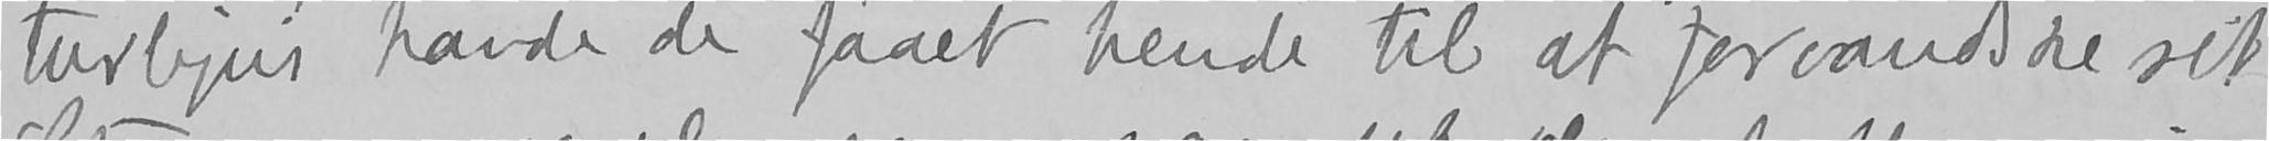turligvis havde de faaet hende til at forvandske sit
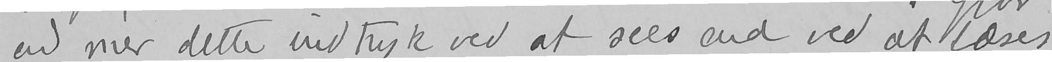end mer dette indtryk ved at sees end ved at læses,
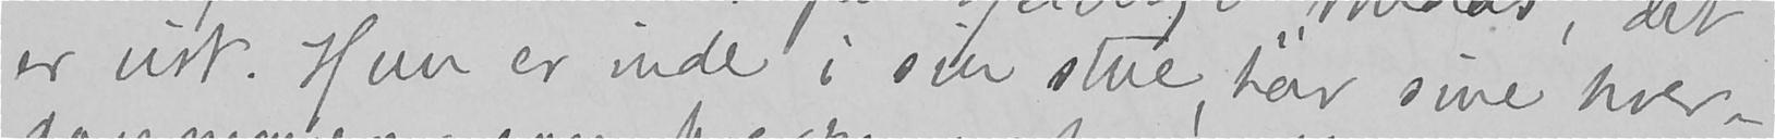er vist. Hun er inde i sin stue, har sine hver-
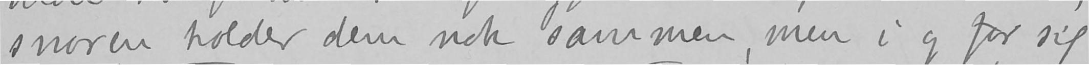snoren holder dem nok sammen, men i og for sig
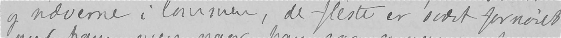og næverne i lommen, de fleste er svært fornøiet
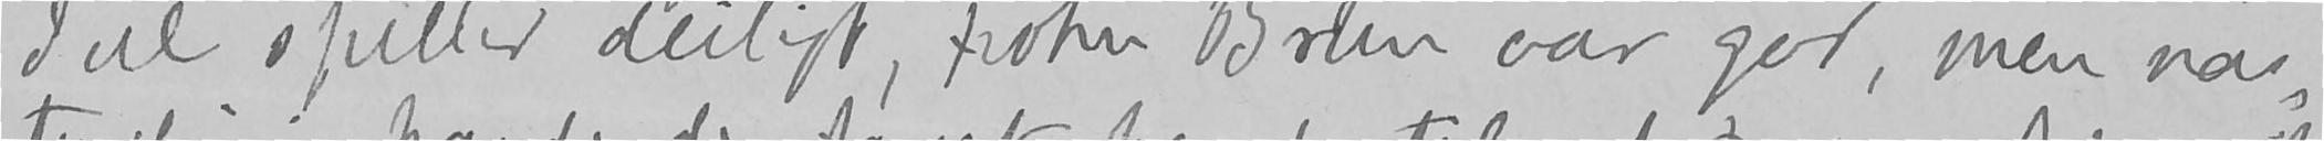Jul spiller deiligt, frøken Brien var god, men na-
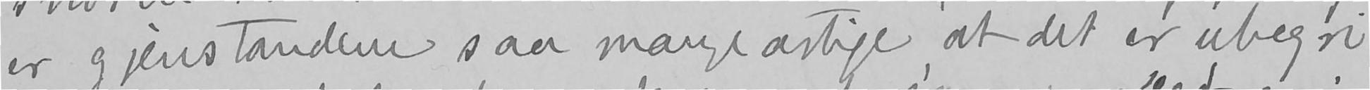er gjenstandene saa mangeartige, at det er ubegri-
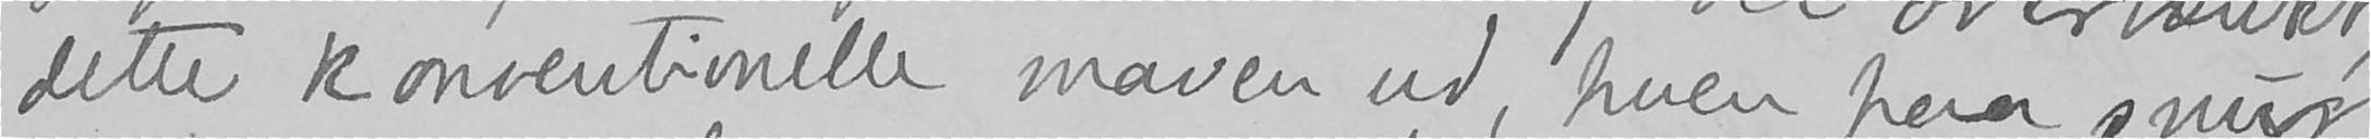dette konventionelle maven ud, huen paa snur
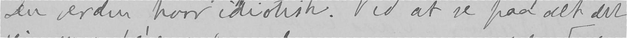Du verden, hvor idiotisk. Ved at se paa alt det
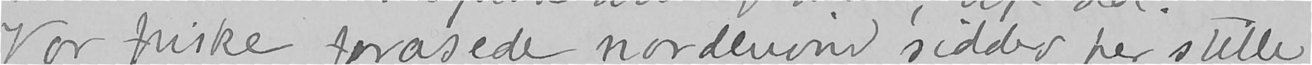Vor friske forasede nordenvind sidder her stille,
